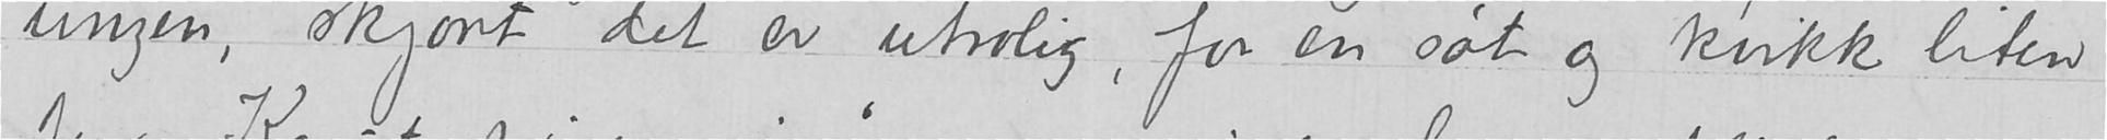ungen, skjønt det er utrolig, for en søt og kvikk liten
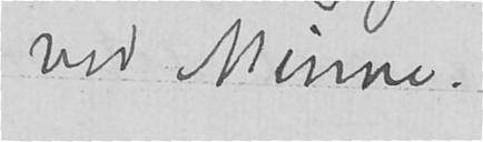vid. Minne.
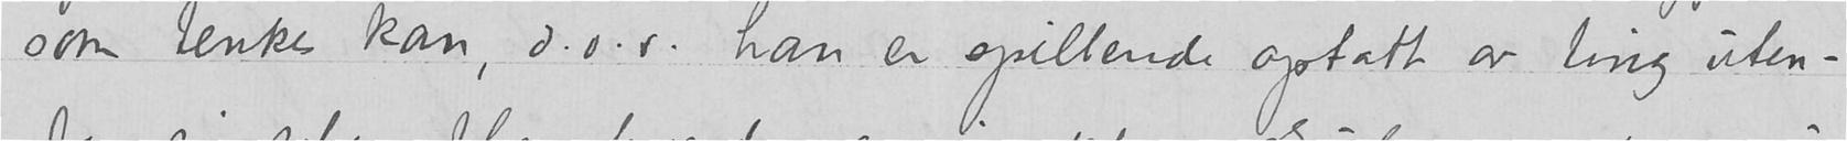som tenkes kan, d.v.s. han er spillende optatt av ting uten-
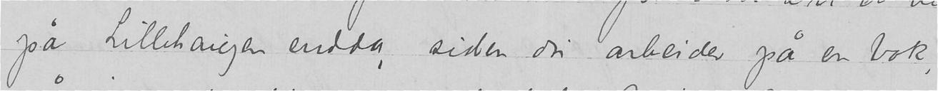pa Lillehaugen endda, siden du arbeider på en bok,
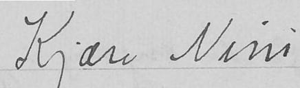Kjære Nini,
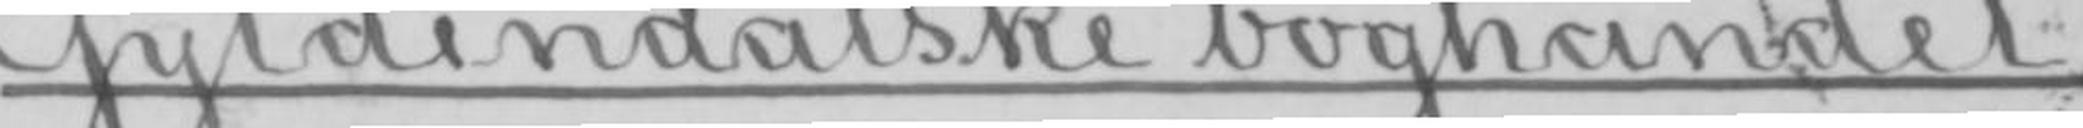Gyldendalske boghandel.
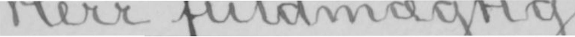Herr fuldmægtig
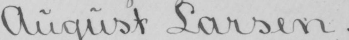August Larsen.
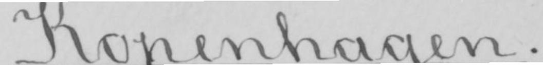Kopenhagen.
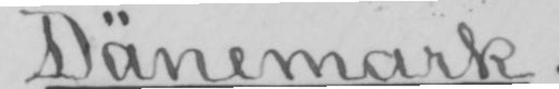Dänemark.
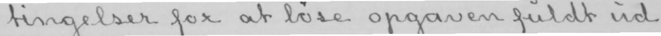tingelser for at løse opgaven fuldt ud
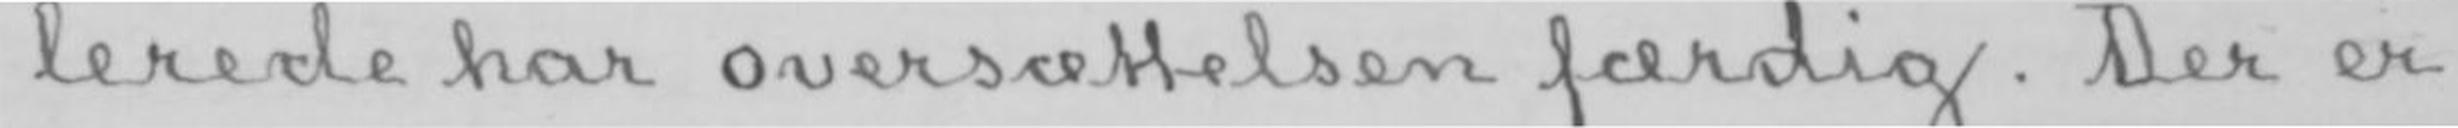lerede har oversættelsen færdig. Der er
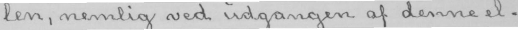len, nemlig ved udgangen af denne el-
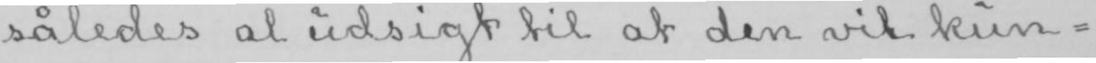således al udsigt til at den vil kun-
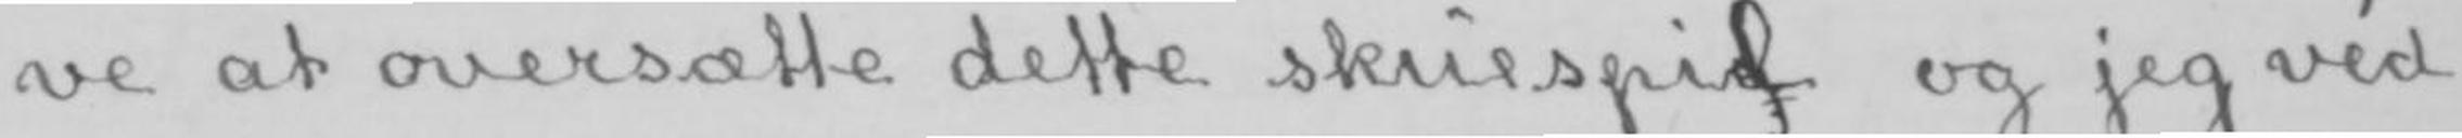ve at oversætte dette skuespigl og jeg véd
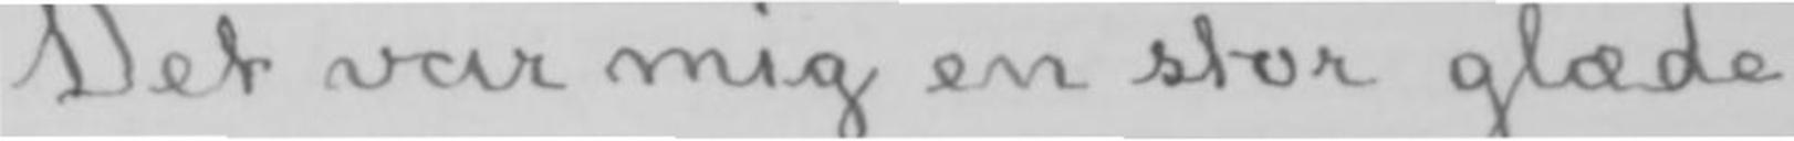Det var mig en stor glæde
